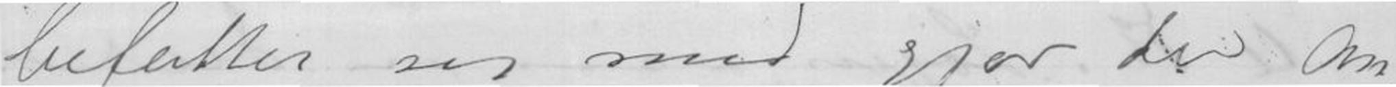befatter sig med gjør den An-
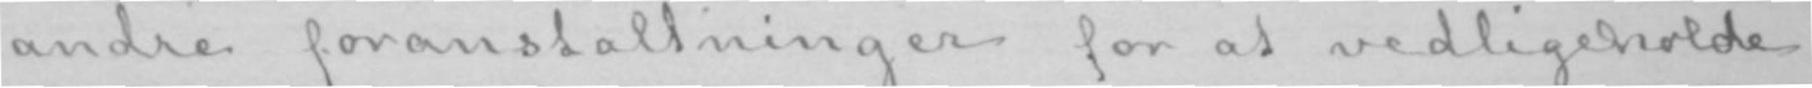andre foranstaltninger for at vedligeholde
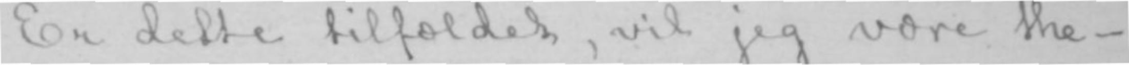Er dette tilfældet, vil jeg være the-
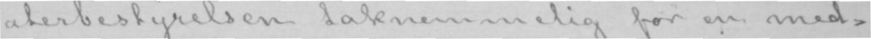aterbestyrelsen taknemmelig for en med-
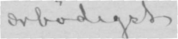ærbødigst
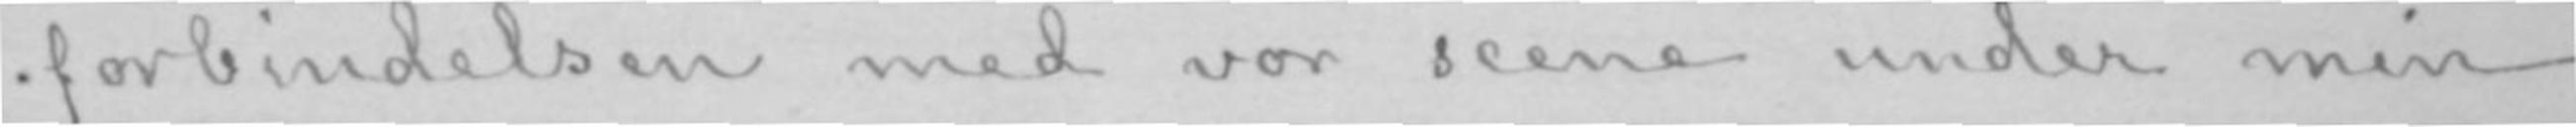forbindelsen med vor scene under min
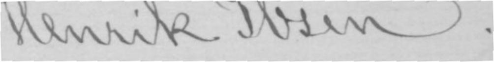Henrik Ibsen.
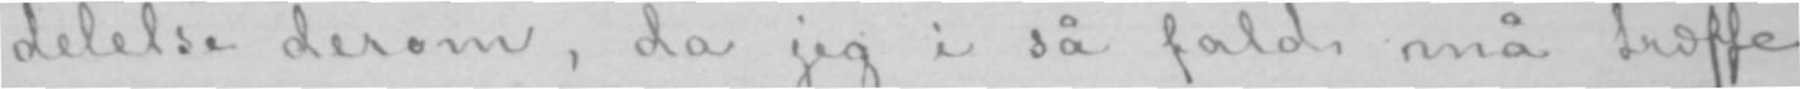delelse derom, da jeg i så fald må træffe
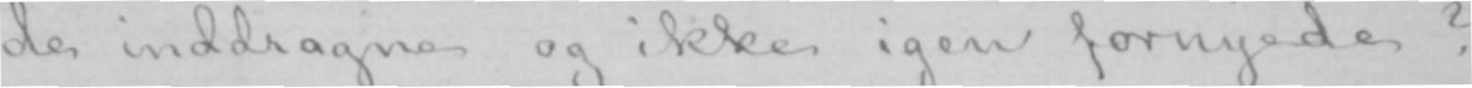de inddragne og ikke igen fornyede?
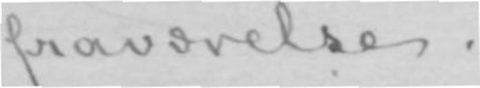fraværelse.
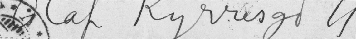Olaf Kyrres gd 11
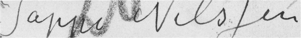Jappe Nilssen
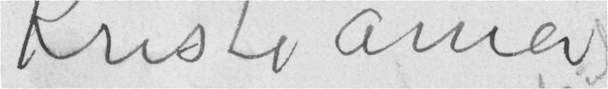Kristiania
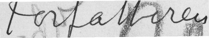Forfatteren
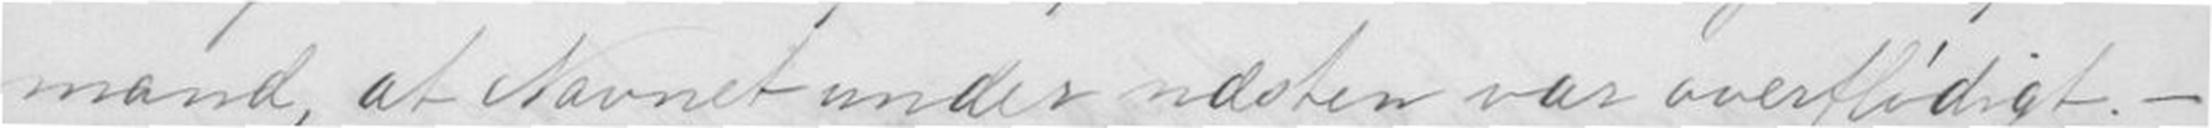mand at Navnet under næsten var overflødigt.-
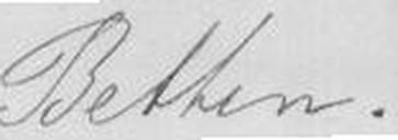Betten.
